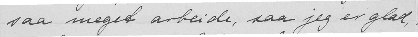saa meget arbeide, saa jeg er glad,
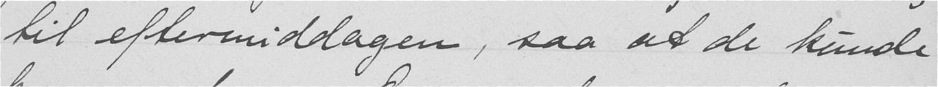til eftermiddagen, saa at de kunde
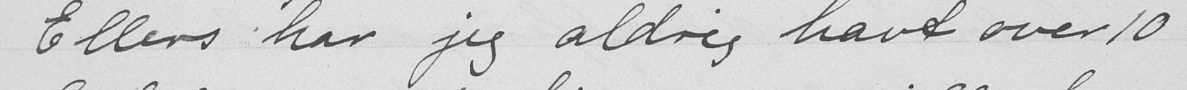Ellers har jeg aldrig havt over 10
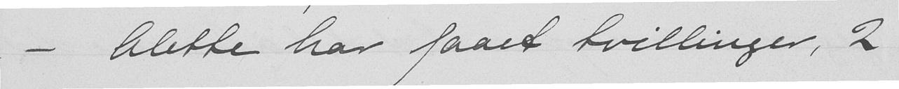- Alette har faaet tvillinger, 2
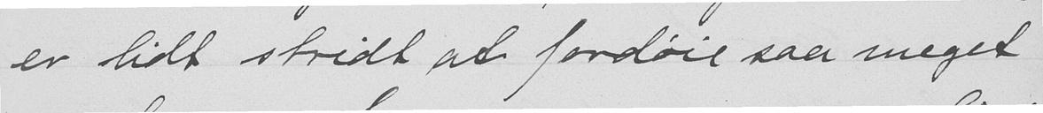er lidt stridt at fordøie saa meget
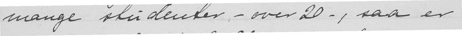mange studenter, - over 20 -, saa er
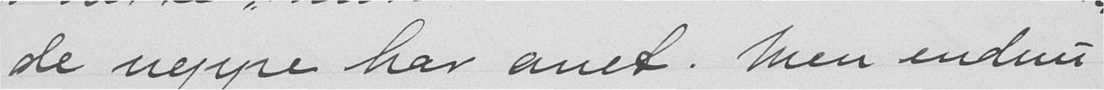de neppe har anet. Men endnu
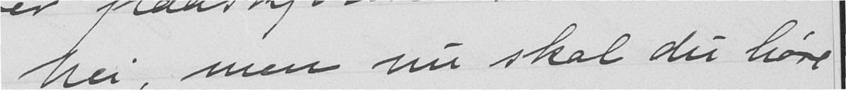- Nei, men nu skal du høre
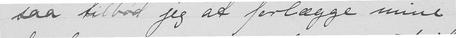saa tilbød jeg at forlægge mine
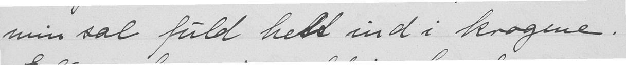min sal fuld helt ind i krogene.
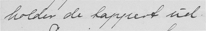holder de tappert ud.
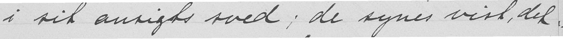i sit ansigts sved; de synes vist, det
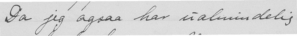Da jeg ogsaa har ualmindelig
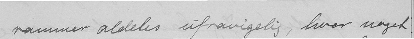rammer aldeles ufravigelig, hvor noget
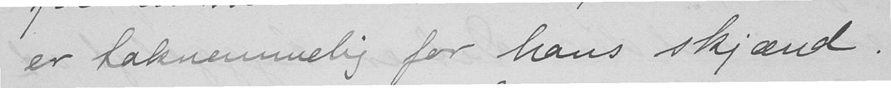er taknemmelig for hans skjænd.
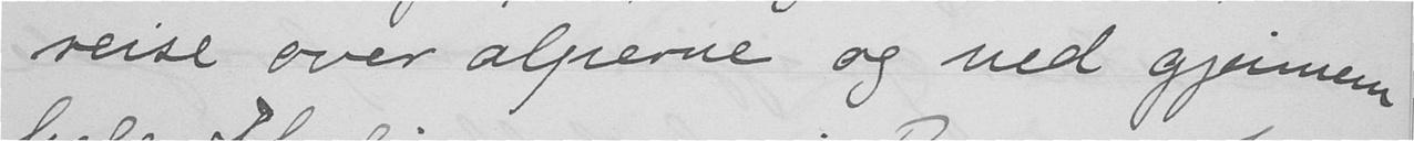reise over alperne og ned gjennem
